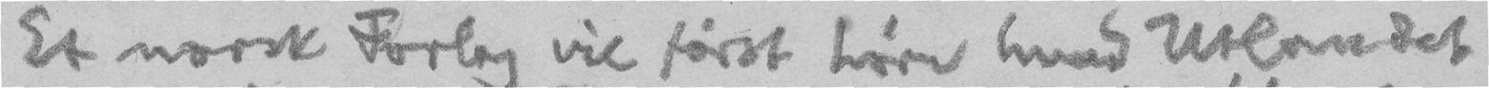Et norsk Forlag vil først høre hvad Utlandet
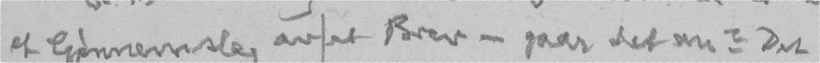et Gjennemslag av|et Brev - gaar det an? Det
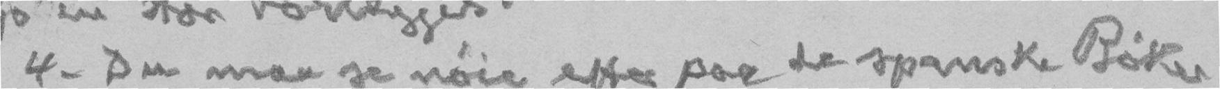4. Du maa se nøie efter paa de spanske Bøker
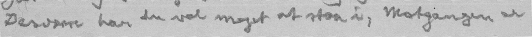Desværre har du vel meget at staa i, Motgangen er
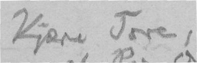Kjære Tore,
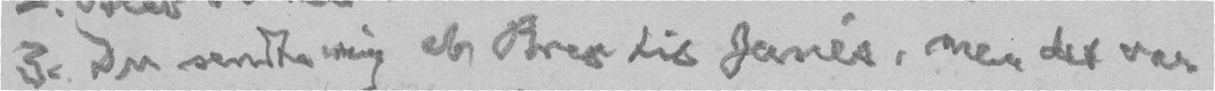3. Du sendte mig et Brev til Janés, men det var
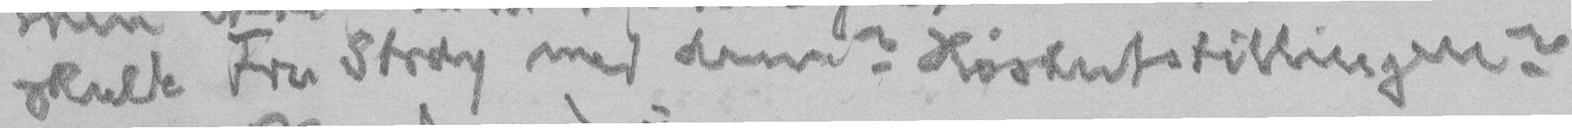skulde Fru Stray med dem? Høstutstillingen?
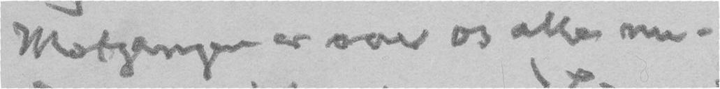Motgangen er over os alle nu.
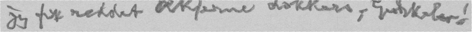jeg fik reddet Aksjerne dokkers, Gudskelov!
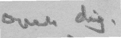over dig.
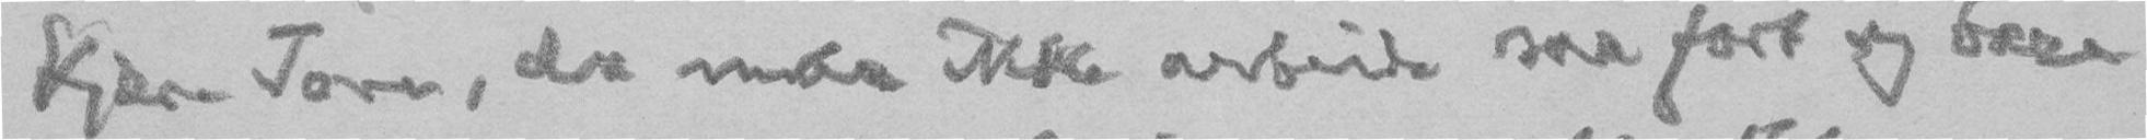Kjære Tore, du maa ikke arbeide saa fort og bare
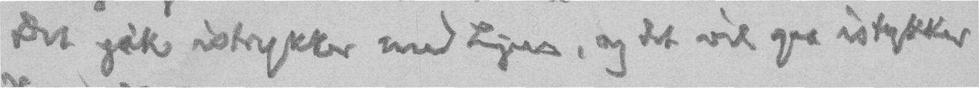Det gik istykker med Ljus, og det vil gaa istykker
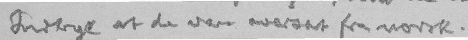Indtryk at de var oversat fra norsk.
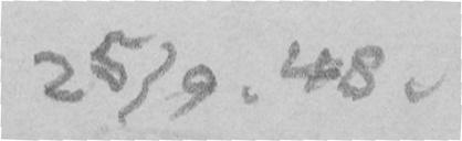25/9. 48.
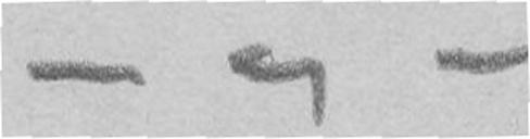- - -
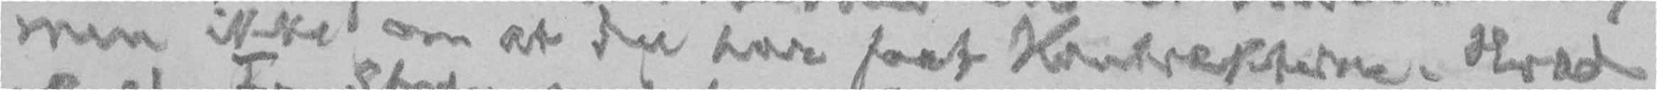men ikke om at du har faat Kontrakterne. Hvad
